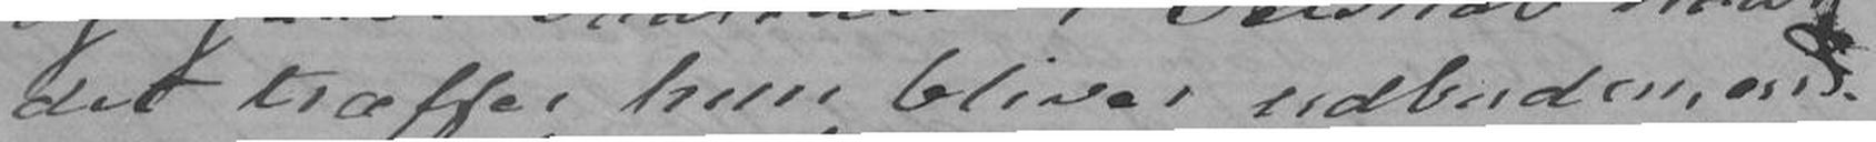det træffes hun bliver udbuden, end-
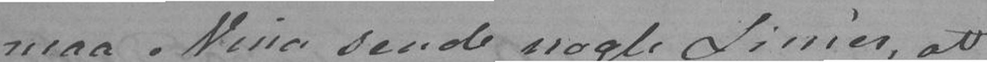maa Nina sende nogle Linier, at
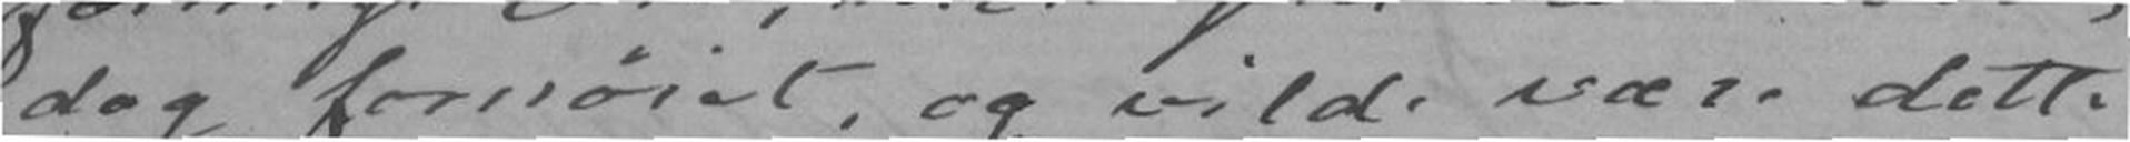dog fornøiet, og vilde være dette
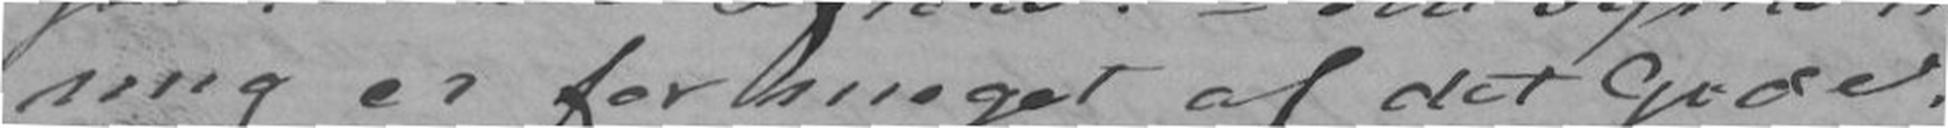mig er formeget af det Gode,
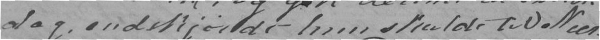dag endskjøndt hun skulde til Neer-
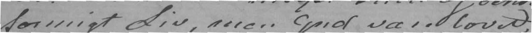formigt Liv, men Gud være lovet,
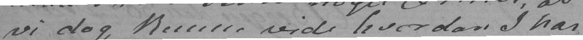vi dog kunne vide hvordan I har
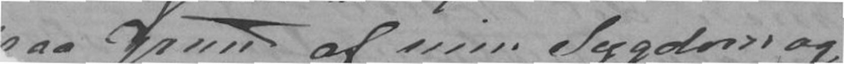aa Grund af min Sygdom og
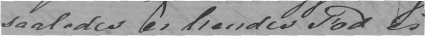saaledes er hendes Fod ei
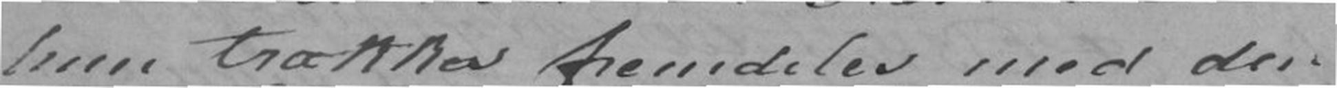hun trækker fremdeles med den
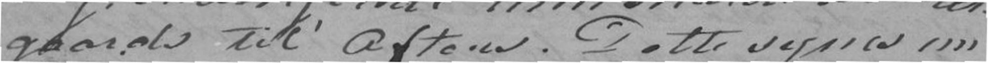gaards til Aftens. Dette synes nu
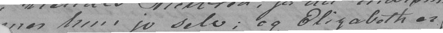mer hun jo selv; og Elizabeth er
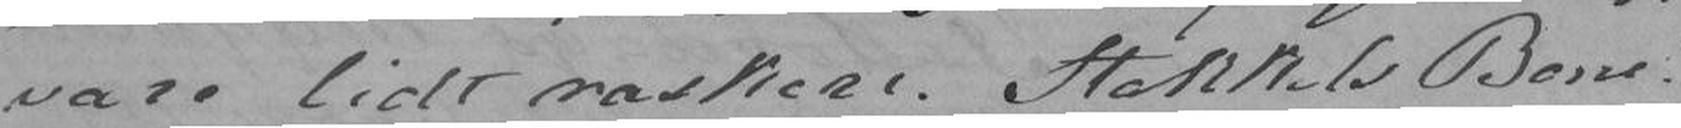vare lidt raskere. Stakkels Bene
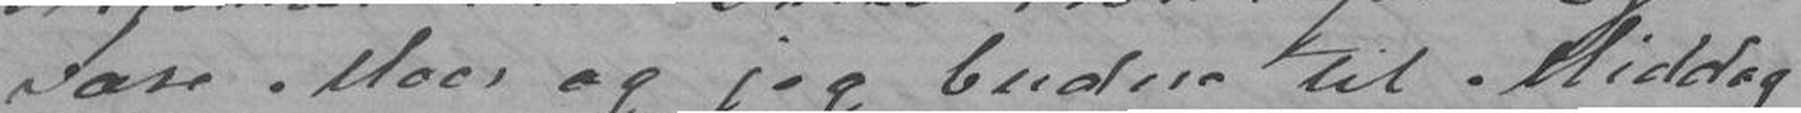vare Moer og jeg buden til Middag
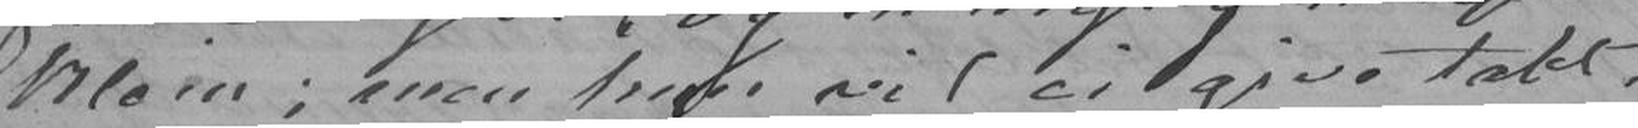klein; men hun vil ei give tabt,
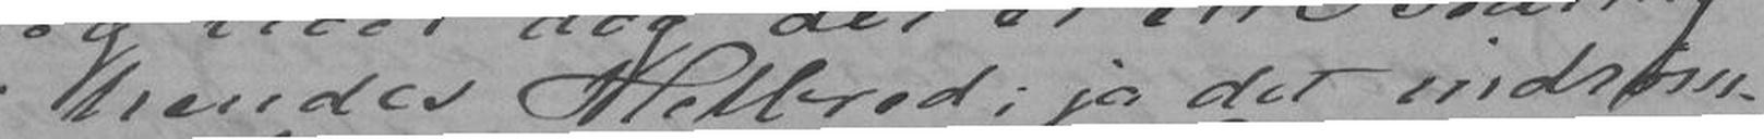i hendes Helbred; ja det indrøm-
